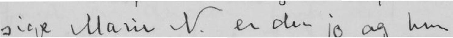sige Marie N. er der jo, og hun
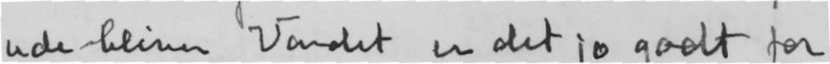udebliver Vandet er det jo godt for
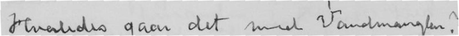Hvorledes gaar det med Vandmangelen?
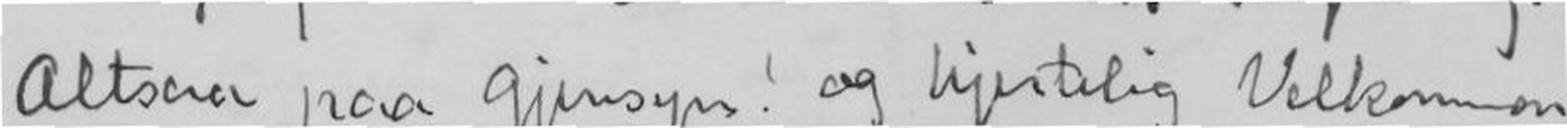Alsaa paa gjensyn! og hjertelig Velkommen.
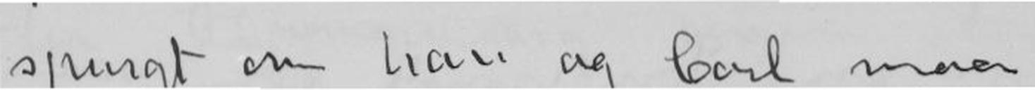spurgt om han og Carl maa
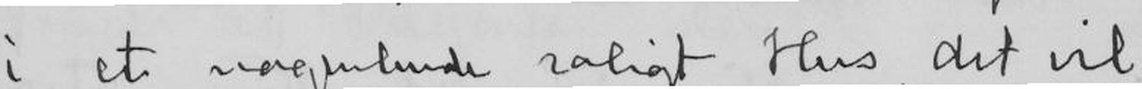i et nogenlunde roligt Hus,det vil
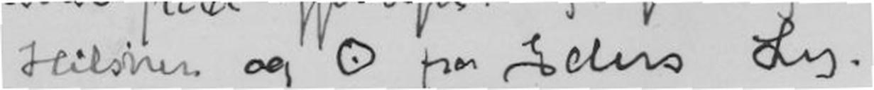Hilsner og 0 fra Eders Ly.
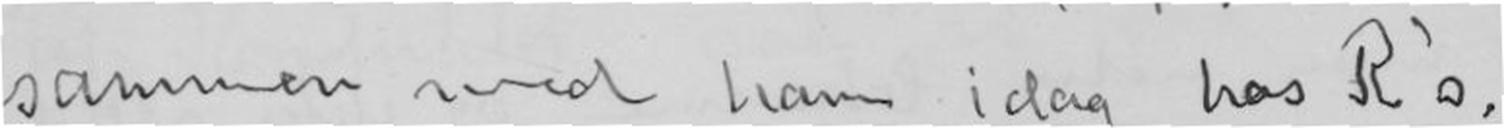sammen med ham idag hos R's.
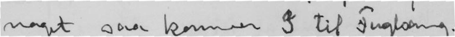noget, saa kommer I til Fuglsang
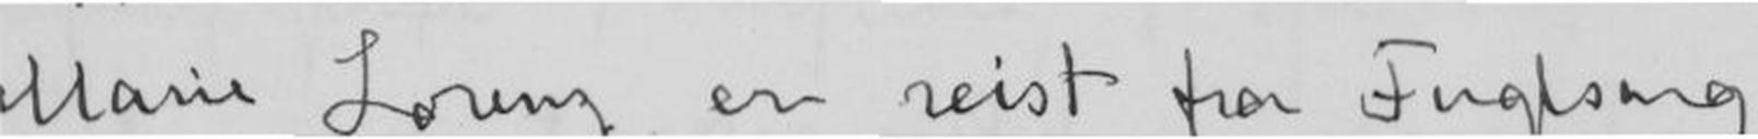Marie Lorenz er reist fra Fuglsang
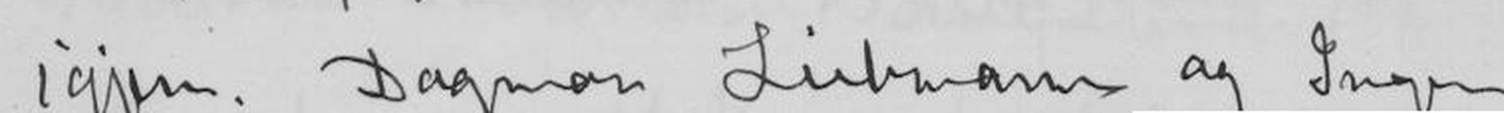igjen. Dagmar Liebman, og Inger
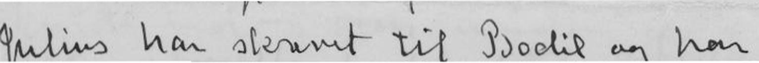Julius har skrevet til Bodil og har
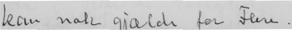kan nok gjælde for Flere.
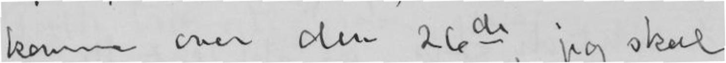komme over den 26de, jeg skal
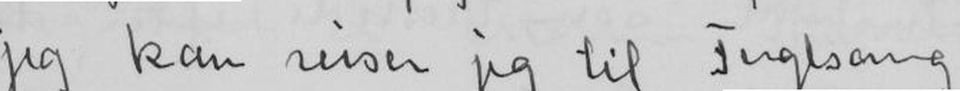jeg kan reiser jeg til Fuglsang
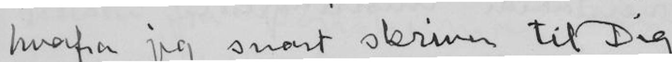hvorfra jeg snart skriver til Dig
